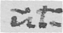ut-
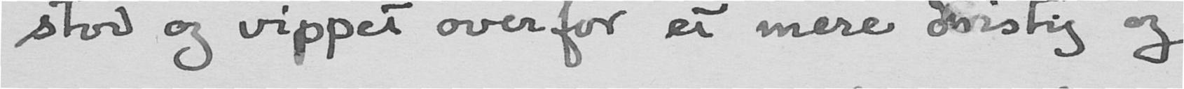stod og vippet overfor et mere dristig og
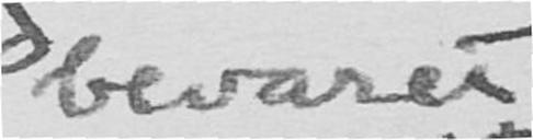bevaret
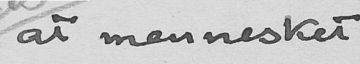at mennesket
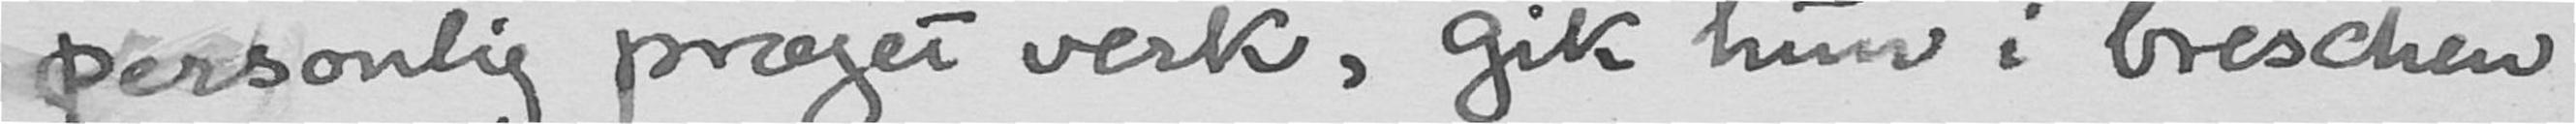personlig præget verk, gik hun i breschen
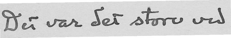Det var det store ved
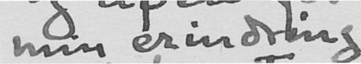min erindring
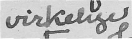virkelige
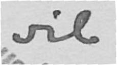vil
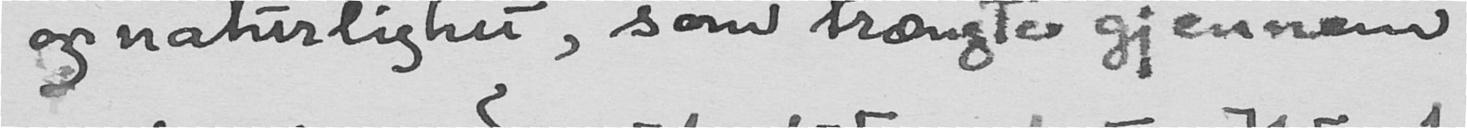og naturlighet, som trængte gjennem
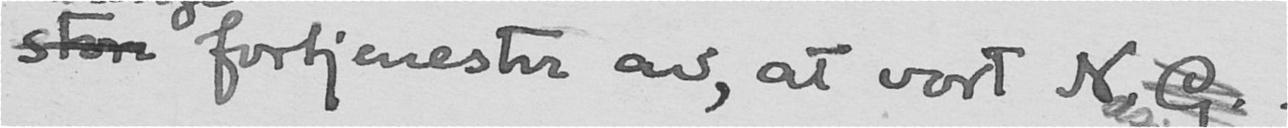store fortjenester av, at vort N.G.
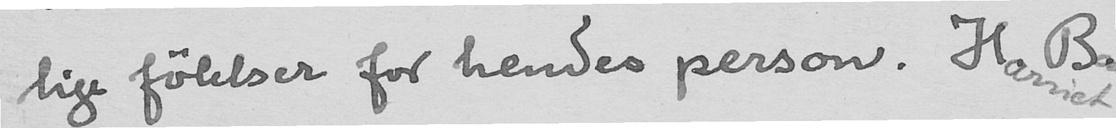lige følelser for hendes person. Harriet
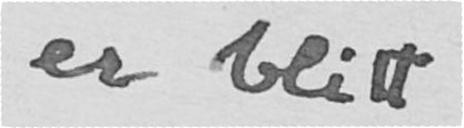er blitt
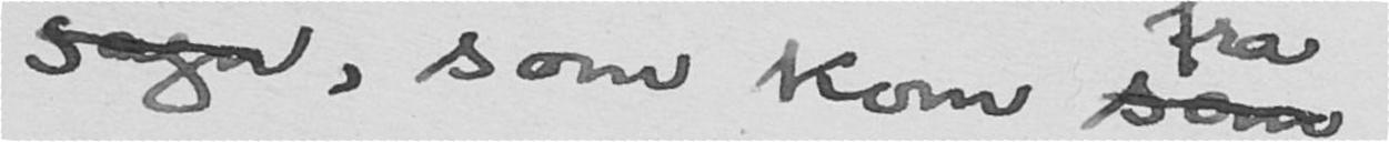sagn, som kom som
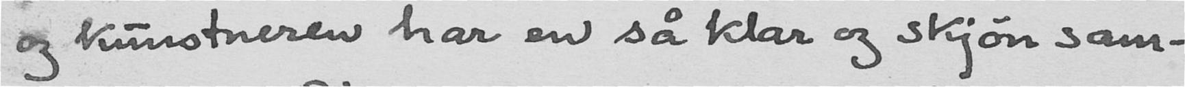og kunstneren har en så klar og skjøn sam-
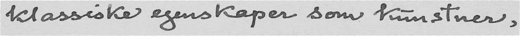klassiske egenskaper som kunstner,
